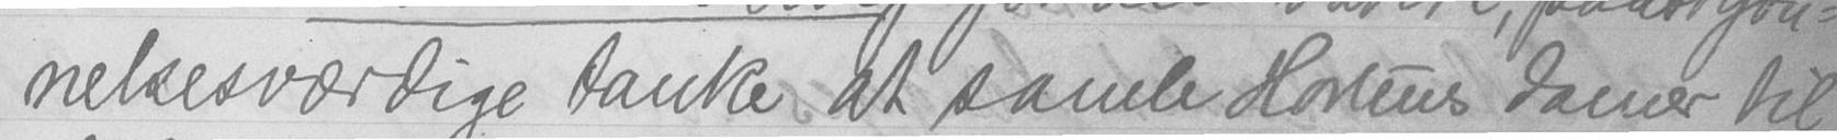nelsesværdige tanke at samle Hortens damer til
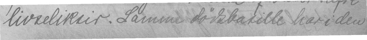livseliksir. Samme dødsbasille har i den
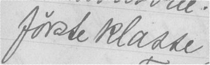første klasse
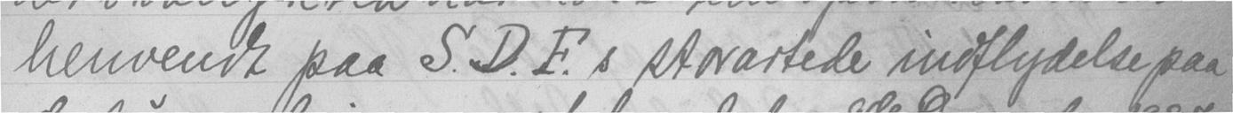henvendt paa S.D.Fs storartede indflydelse paa
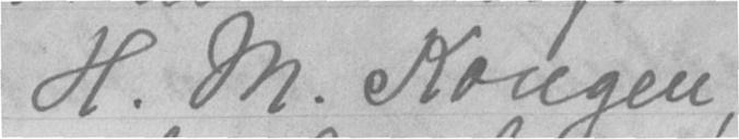H. M. Kongen,
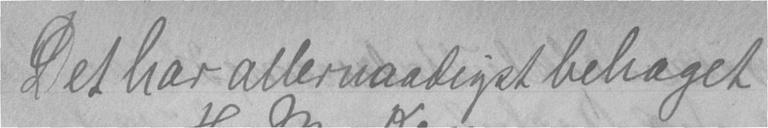Det har allernaadigst behaget
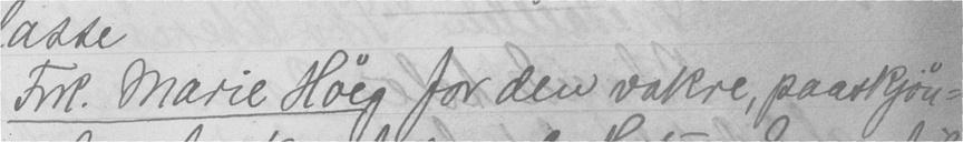Frk. Marie Høeg for den vakre, paaskjøn-
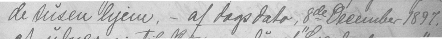de tusen hjem, - af dags dato, 8de December 1897.
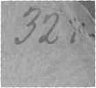32
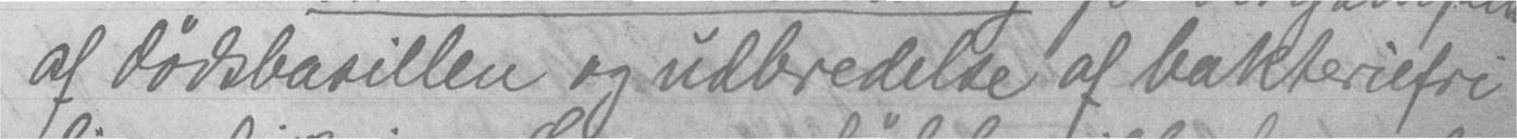af dødsbasillen og udbredelse af bakteriefri
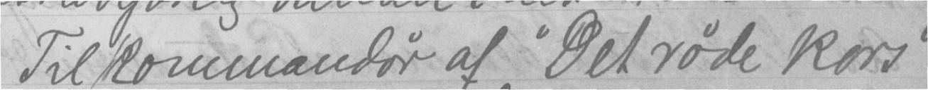Til kommandør af "Det røde kors"
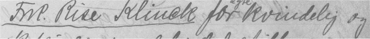Frk. Rise Klinck for ægte kvindelig og
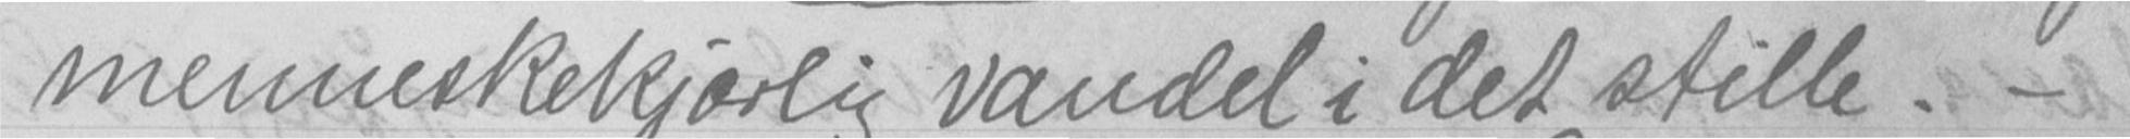menneskekjærlig vandel i det stille. -
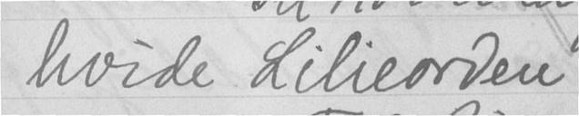hvide Lilieorden"
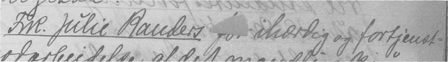Frk. Julie Randers for ihærdig og fortjenst-
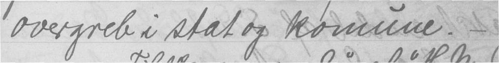overgreb i stat og kommune. -
